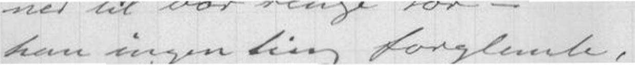han ingen ting forglemte,
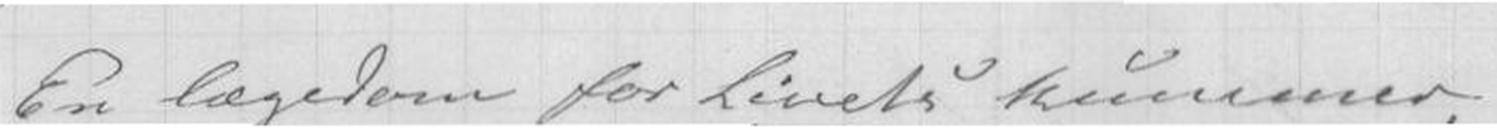En lægedom for livets kummer,
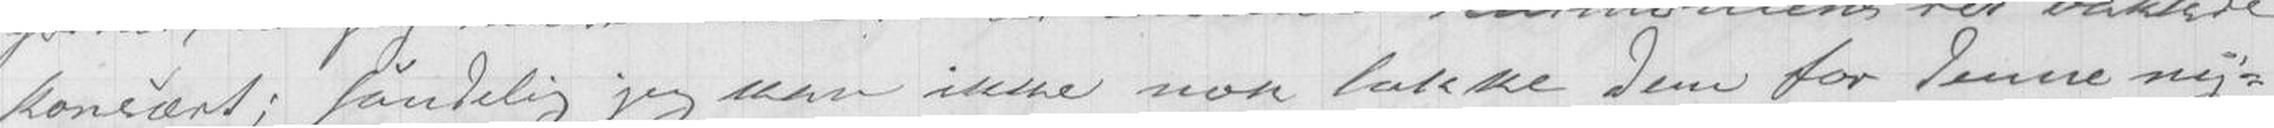konsært; sandelig jeg kan ikke nok takke Dem for Denne ny-
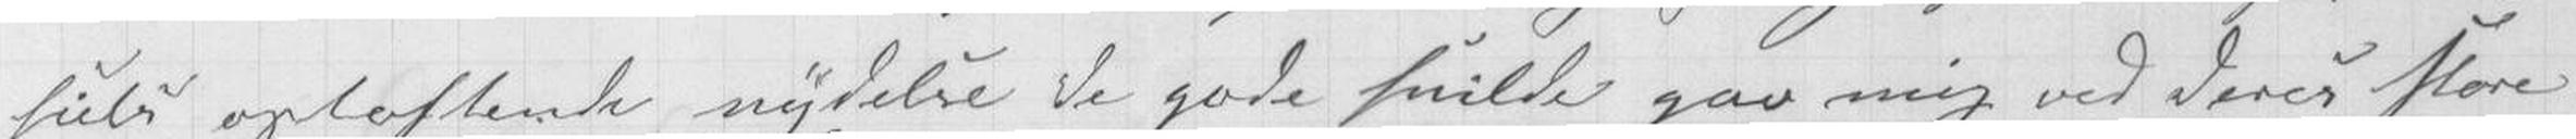siets oploftende nydelse De gode snilde gav mig ved Deres store
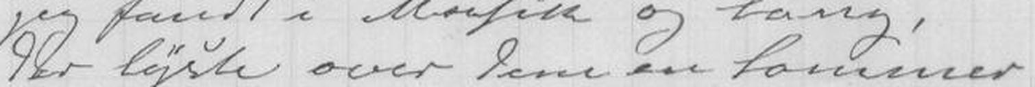Det lyste over Dem en Sommer
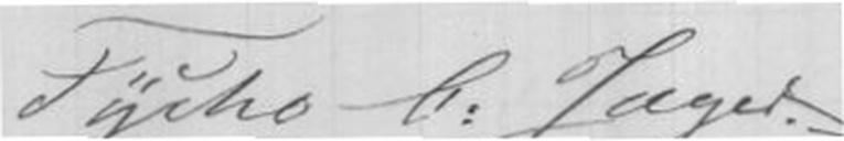Tycho C. Jæger
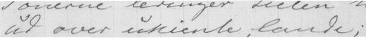ut over ukiente lande;
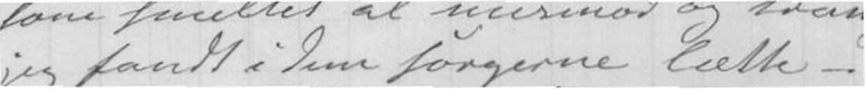jeg fandt i Dem sorgerne lætte -
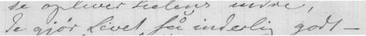De gjør Livet så inderlig godt
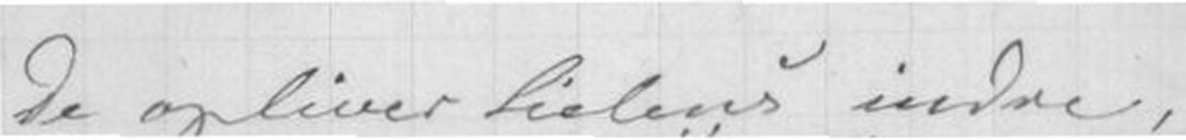De oplever tielens indre,
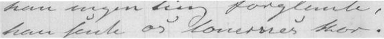han sente os tonernes Kor.
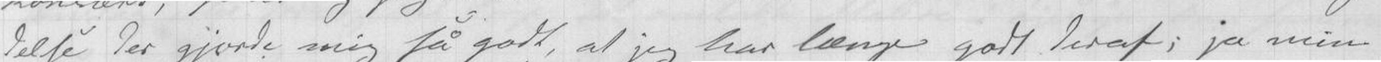telse Der gjorde mig så godt, at jeg har længe godt deraf ja min
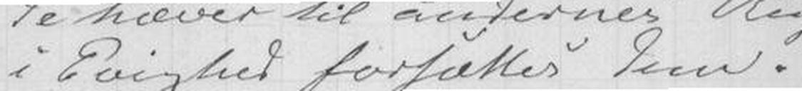i Evighed forsættes Dem.
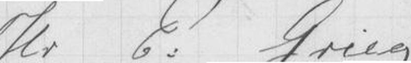Hr. E: Grieg!
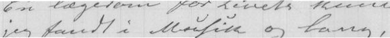jeg fandt i Musik og Sang,
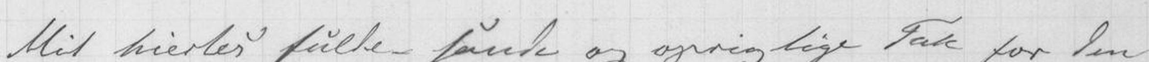Mit hiertes fulle sande og oprigtige Tak for den
